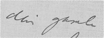din gamle
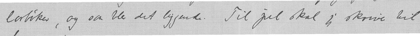lovbøker, og saa ble det liggende. Til jul skal jeg skrive til
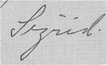Sigrid.
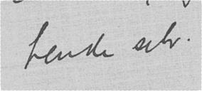hende selv.
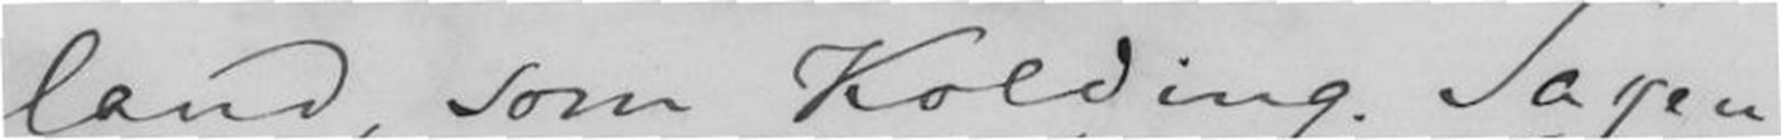land, som Kolding. Sagen
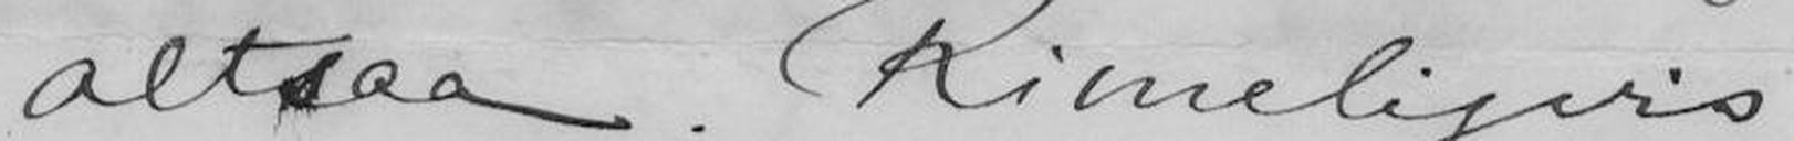altsaa. Rimeligvis
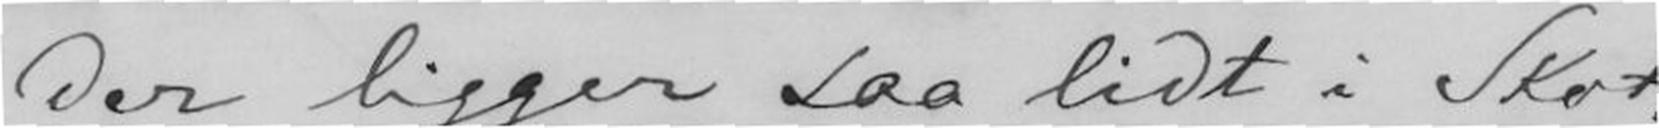der ligger saa lidt i Skot-
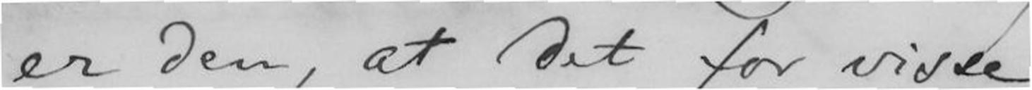er den, at det for visse
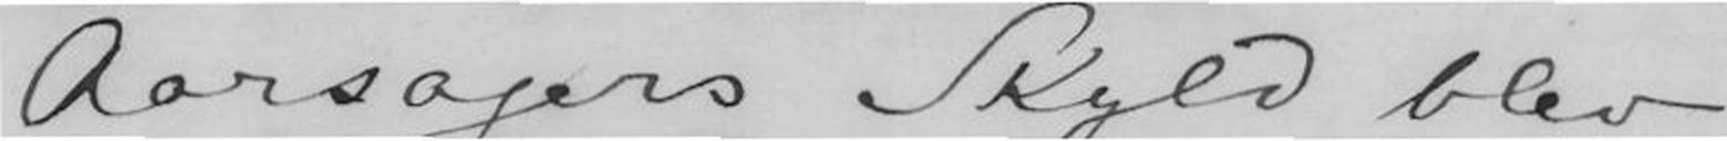Aarsagers Skyld blev
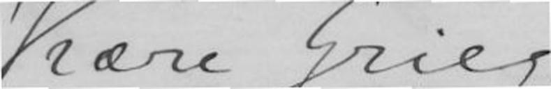Kære Grieg,
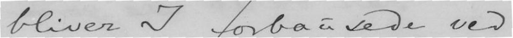bliver I forbausede ved
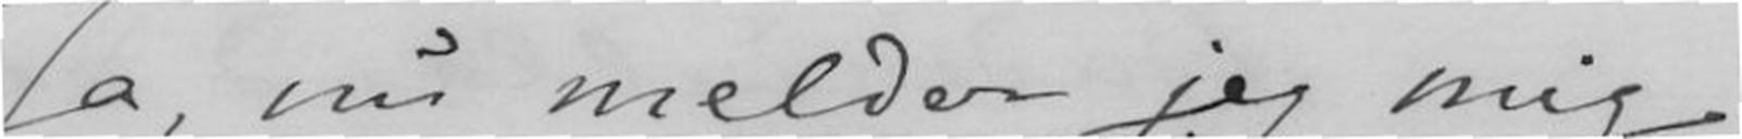Ja, nu melder jeg mig
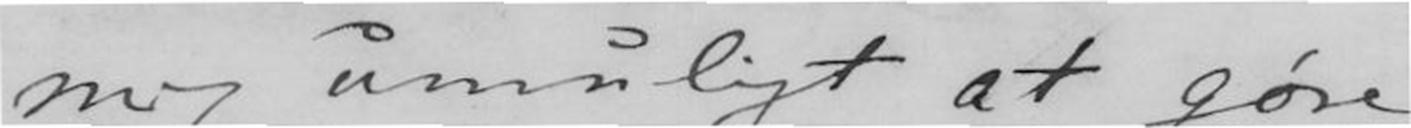mig umuligt at gøre
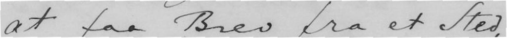at faa Brev fra et Sted
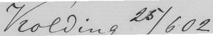Kolding 25/6 02
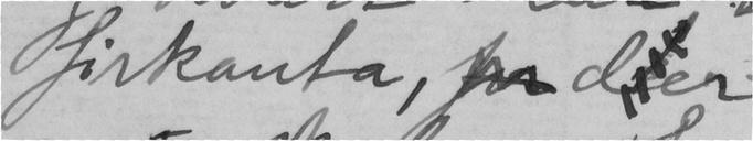firkanta, for der
